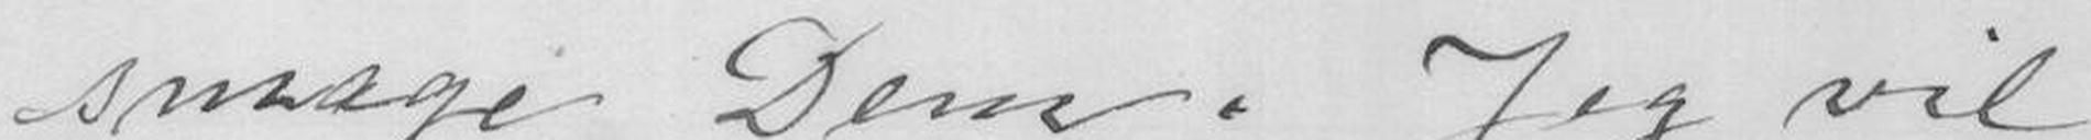smage Dem. Jeg vil
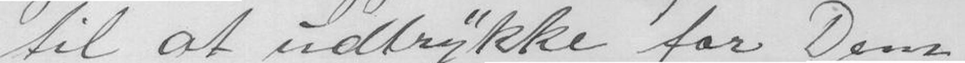til at udtrykke for Dem
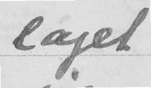laget
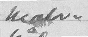Malor-
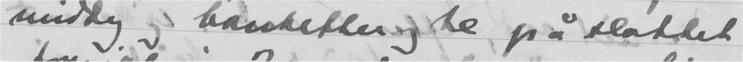middag og banketter også i slatdet
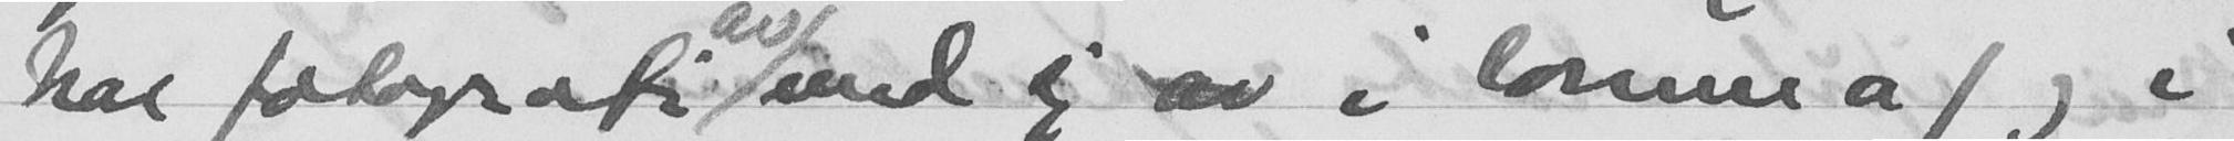har fotografi av med sig i lomma) og i
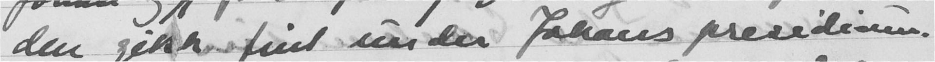den gikk fint under Johans presidium.
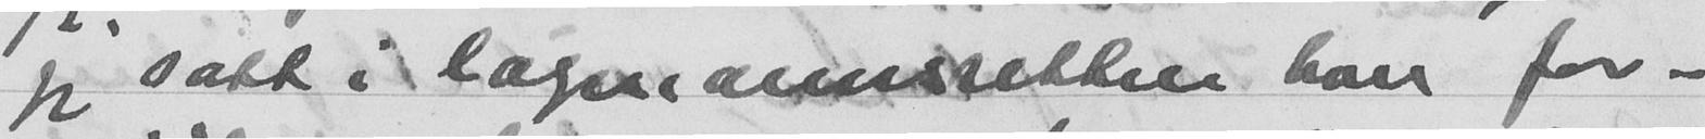jeg satt i lagnianunsitten hver for-
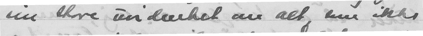sin store undenket om alt som ikke
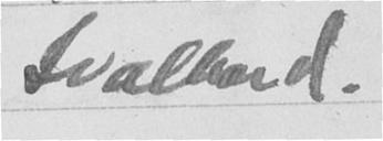Svalbard.
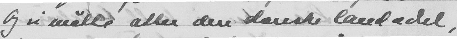Og vi møtte atter den danske landadel,
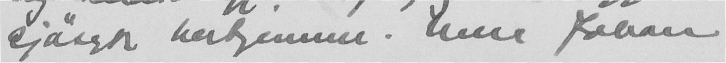sjøsyk herhjemme. Men Johan
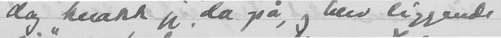dag knakk jeg da også, og blev liggende
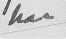har
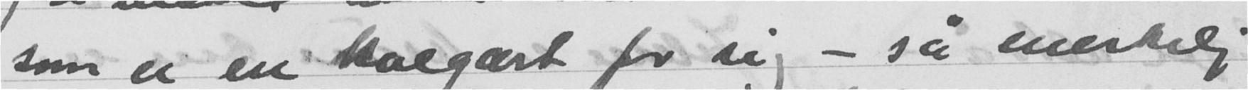som er en kvegart for sig - så merkelig
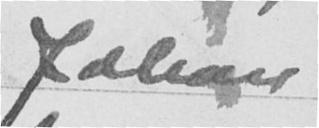Johan
